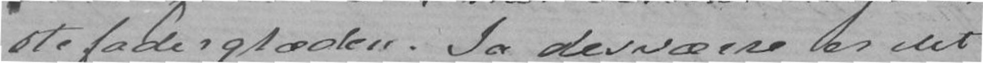stefaderglæden. Ja desværre er det
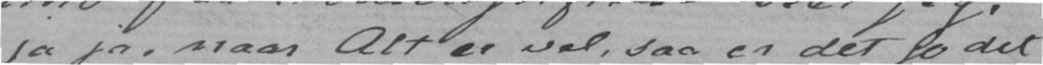ja ja, naar Alt er vel, saa er det jo det
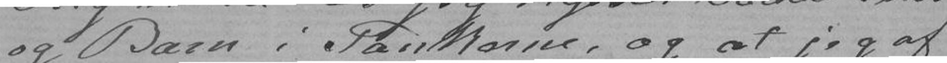og Barn i Tankerne, og at jeg af
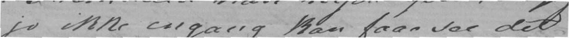jo ikke engang kan faae see det
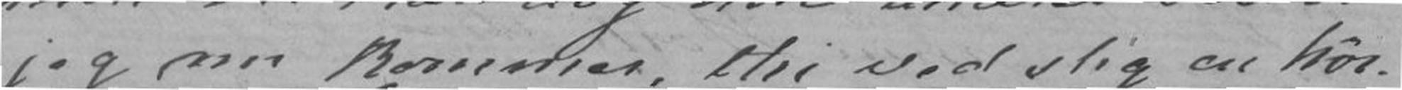jeg nu kommer, thi ved slig en høi-
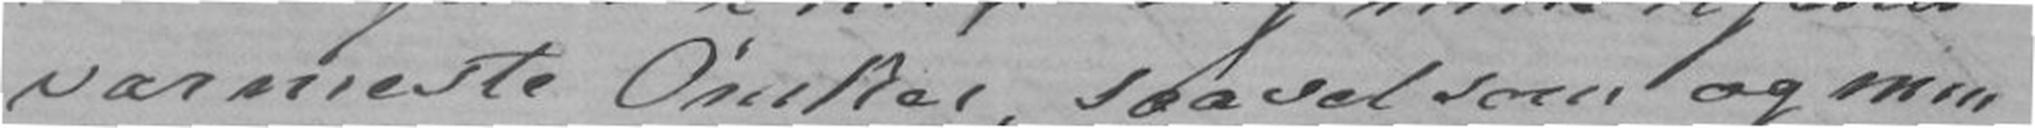varmeste Ønsker, saa selsom og min
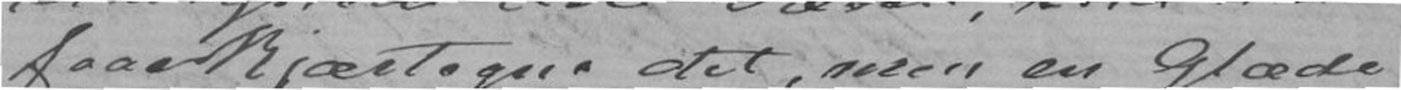faaer kjærtegne det, men en Glæde
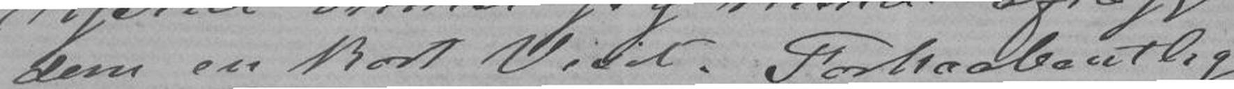dem en kort Visit. Forhaabentlig
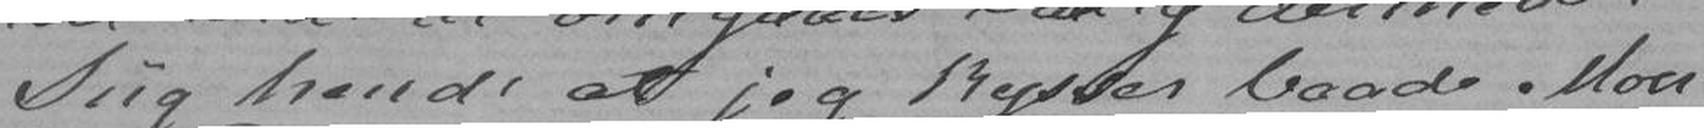Siig hende at jeg kysser baade Moer
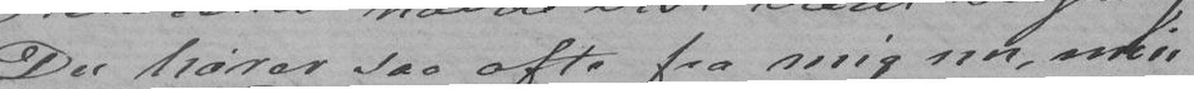Du hører saa ofte fra mig nu, min
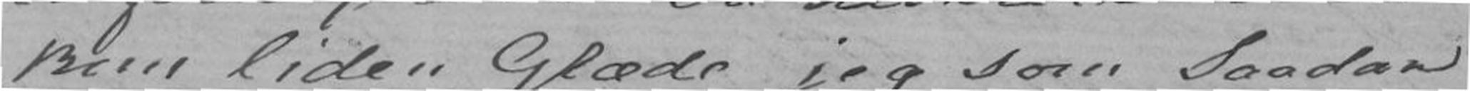kun liden Glæde jeg som saadan
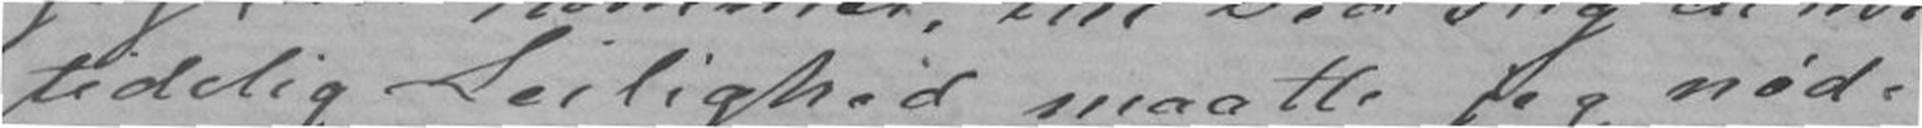tidelig Leilighed maatte jeg nød-
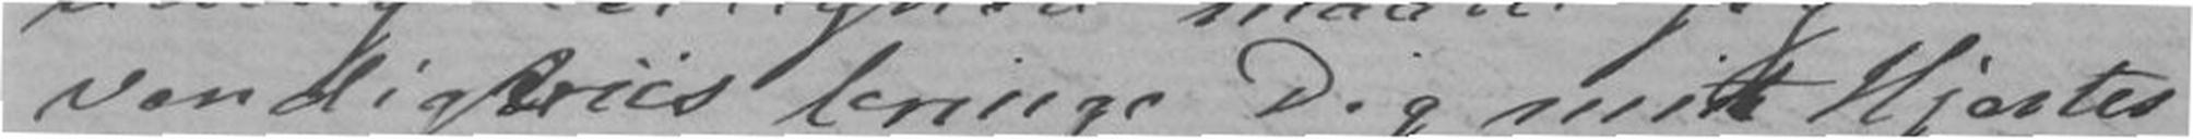vendigviis bruge Dig mit Hjertes
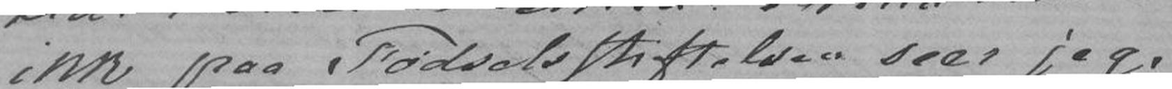ikke paa Fødselsstiftelsen seer jeg,
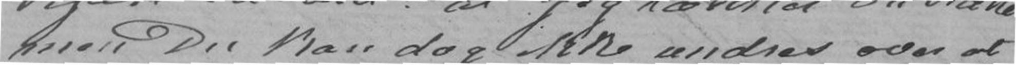men Du kan dog ikke undres over at
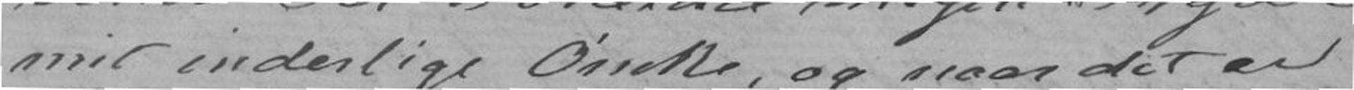mit inderlige Ønske, og naar det er
